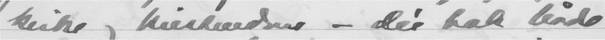kirke og kristendom - der bak både
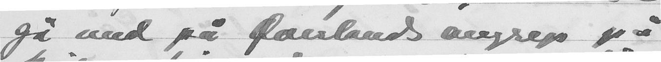gå med på Øverlands angrep på
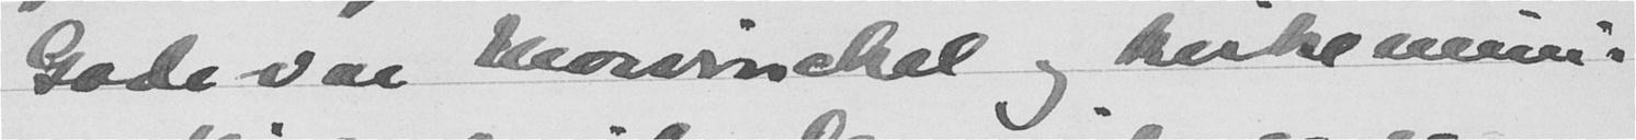Gode var Mowvinckel og kirkemini-
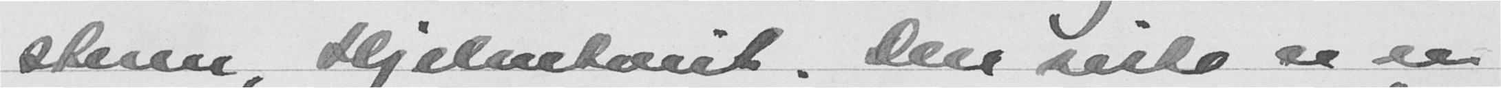steren, Hjelmtveit. Den siste er en
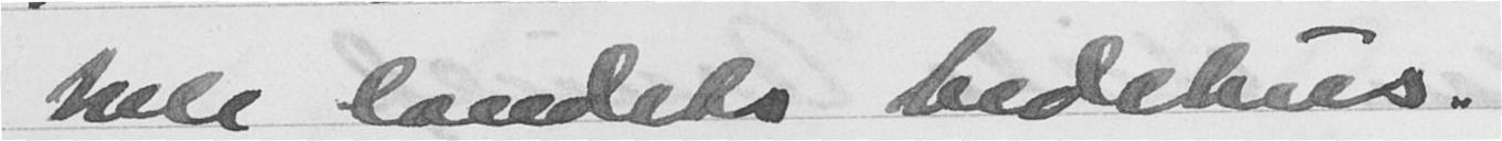hele landets bedehus.
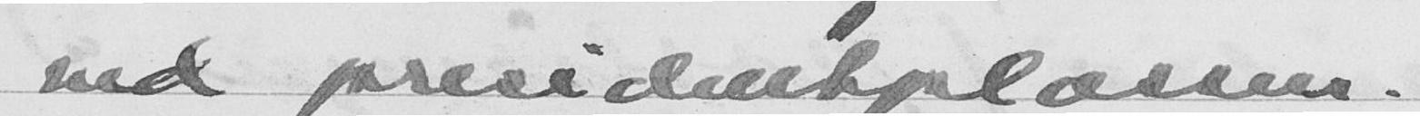ved presidentplassen.
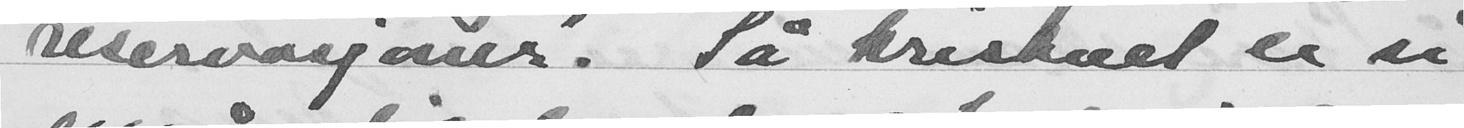reservasjoner. Så kristnet er vi
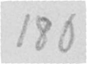180
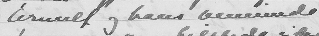Arnulf og hans blivende
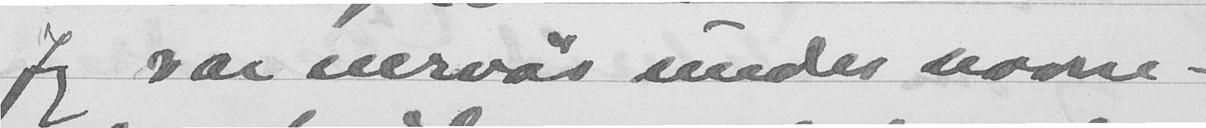Jeg var nervøs under navne-
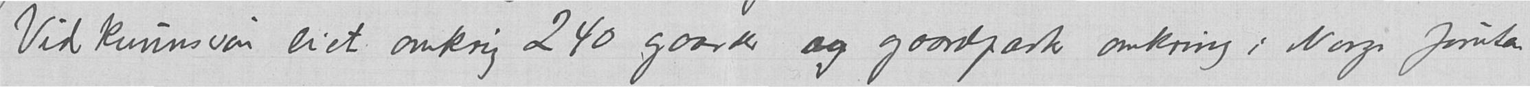Vidkunnsson eiet omkring 240 gaarder og gaardpark omkring i Norge foruten
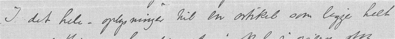I det hele – oplysninger til en artikel som ligger helt
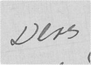Deres
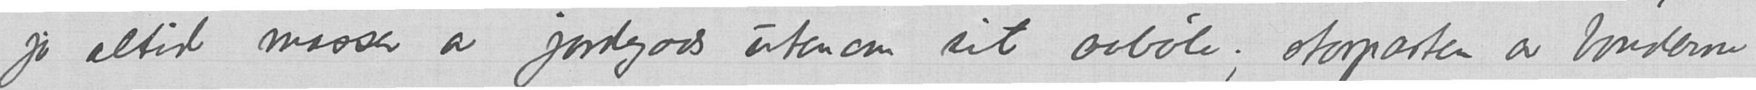jo altid masser av jordegods utenom sit aabøle; storparten av bønderne
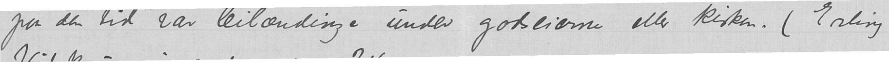paa den tid var leilændinge under godseierne eller kirken. (Erling
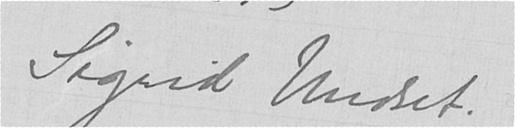Sigrid Undset.
